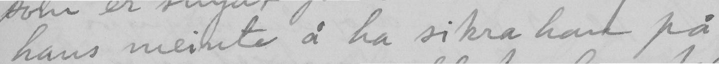hans meinte å ha sikra han på
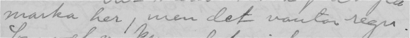marka her, men det vantar regn.
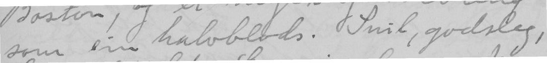som ein halvblods. Snil, godsleg,
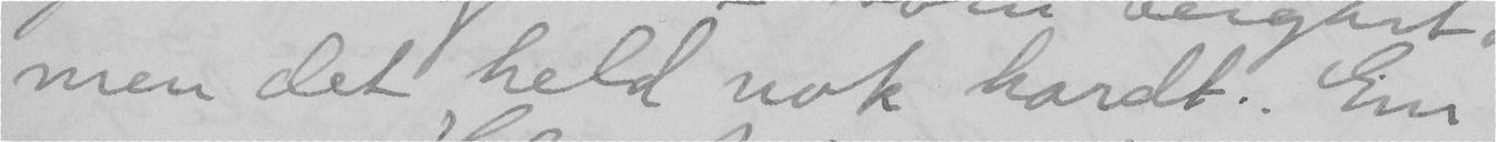men det held nok hardt. Ein
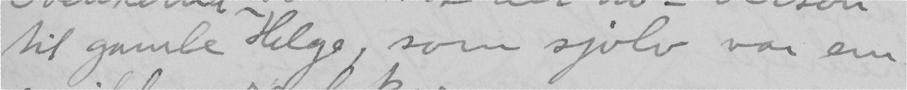til gamle Helge, som sjölv var ein
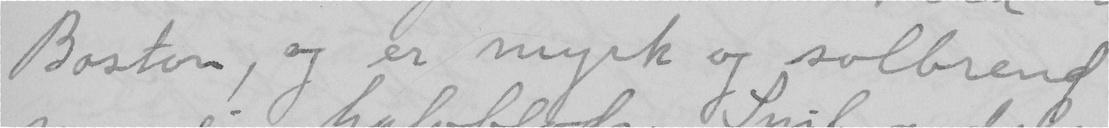Boston, og er myrk og solbrend
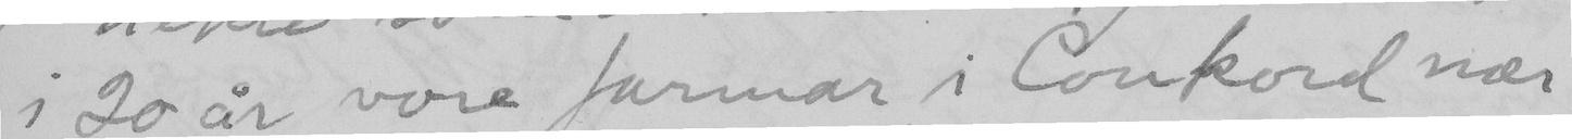i 20 år vore farmar i Conkord nær
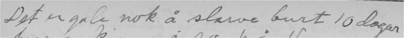Det er gale nok å slarve burt 10 dagar
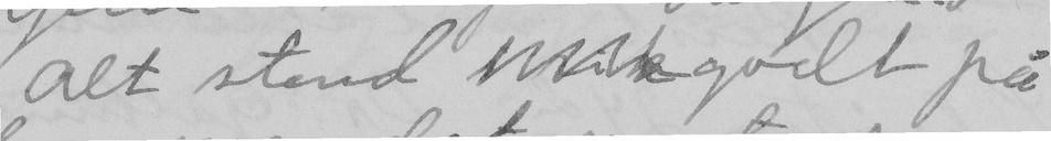Alt stend  godt på
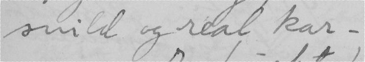snild og real kar –
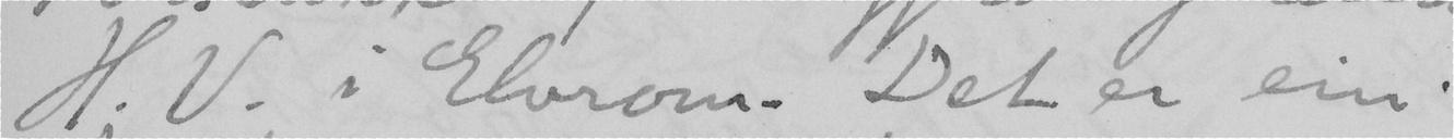H.V. i Elvrom. Det er ein
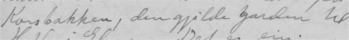Korsbakken, den gjilde garden til
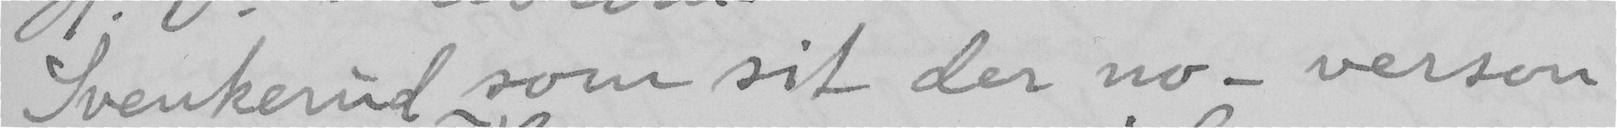Svenkerud som sit der no – verson
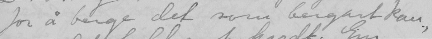for å berge det som bergast kan,
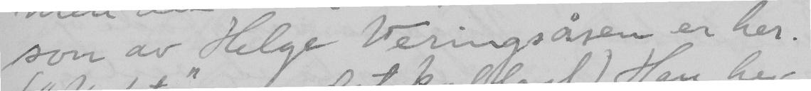son av Helge Veringsåsen er her.
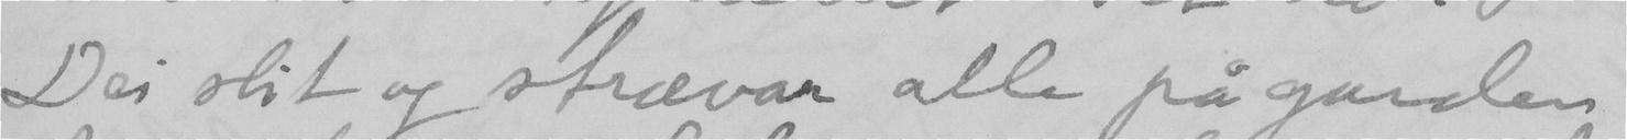Dei slit og strævar alle på garden
